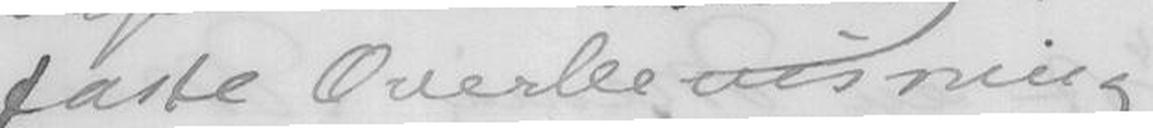faste Overbevisning
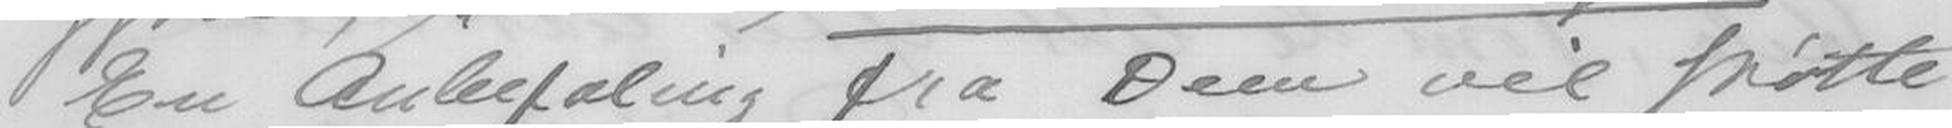En Anbefaling fra Dem vil støtte
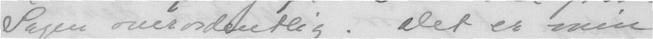Sagen overordentlig. Det er min
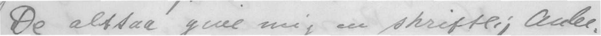De altsaa give mig en skriftlig Andbe-
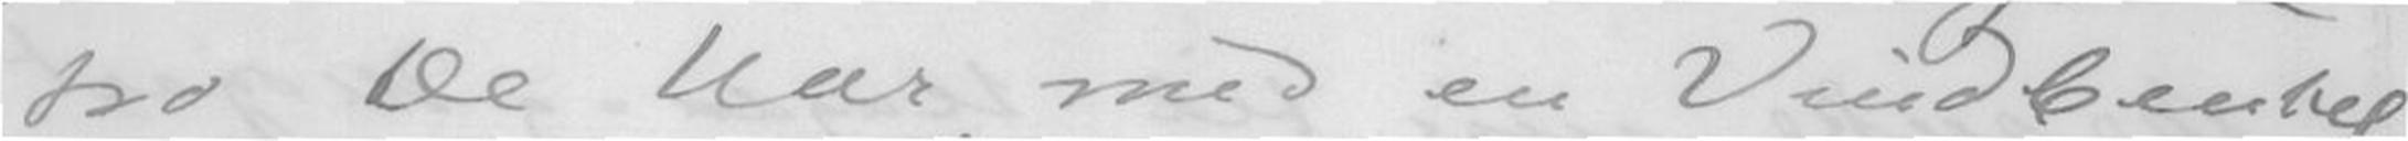tro De har med en Vindbentel
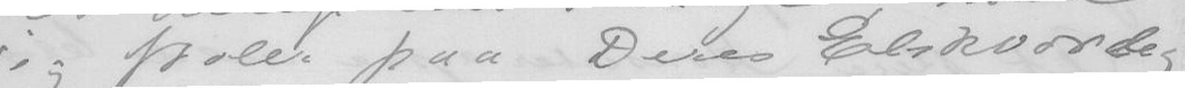jeg stoler paa Deres Elskværdig-
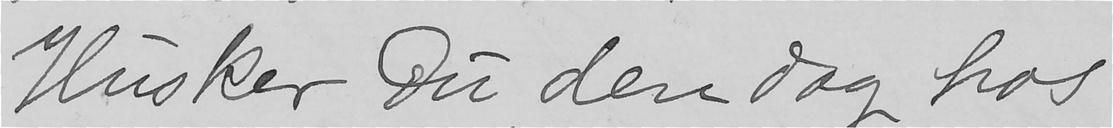Husker Du den dag hos
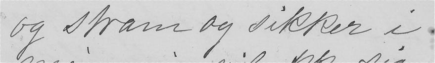og stram og sikker i
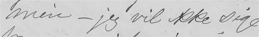min - jeg vil ikke sige
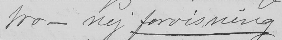tro - nej forvisning
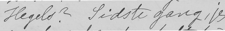Hegels? Sidste gang, jeg
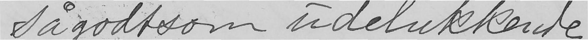sågodtsom udelukkende
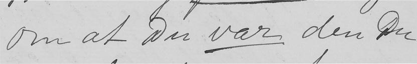om at Du var den Du
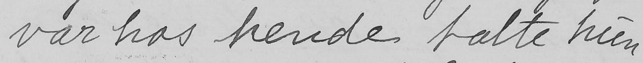var hos hende talte hun
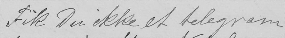Fik Du ikke et telegram
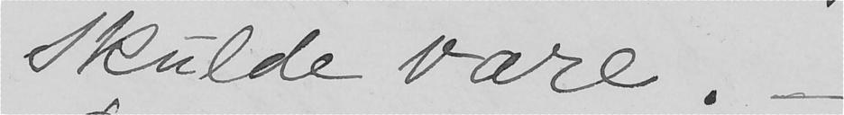skulde være. -
